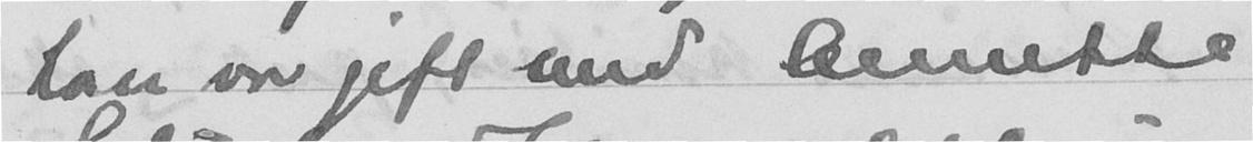han var gift med Anette
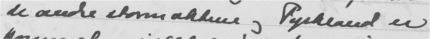de andre stormaktene og Tyskland er
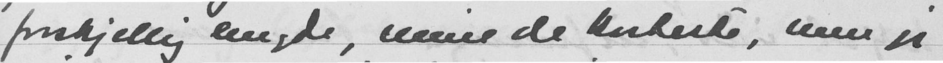forskjellig lengde, mine de korteste, men jeg
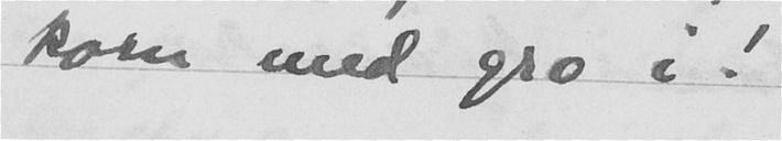roter med gro i:
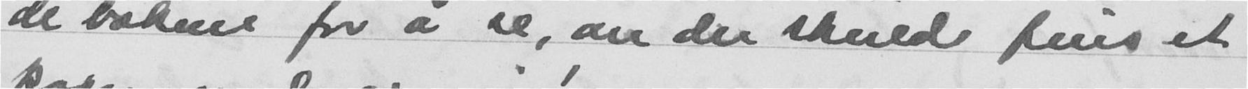de bøkene for å se, om der skulde fins et
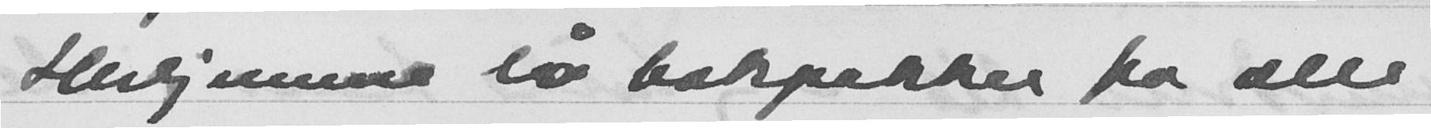Her hjemme lå bokpakker fra alle
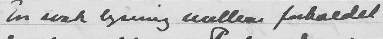En svak lysing mellom forholdet
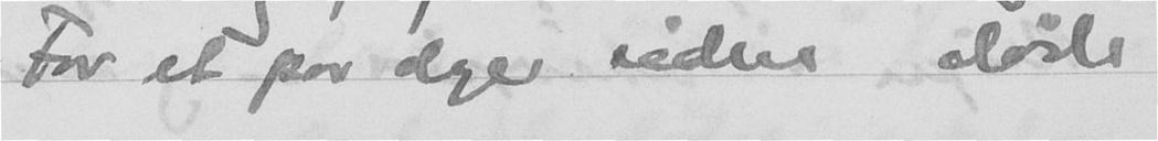For et par dager siden døde
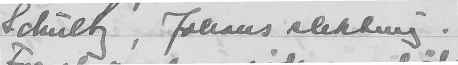Schultz, Johans slekting.
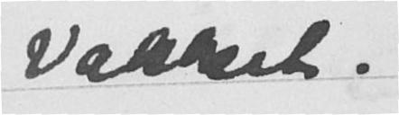vakkert.
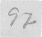97
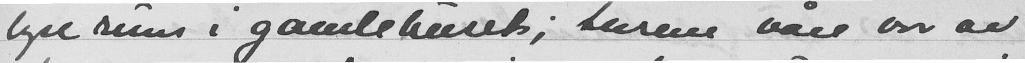lyse rum i gamlehuset; turene våre var av
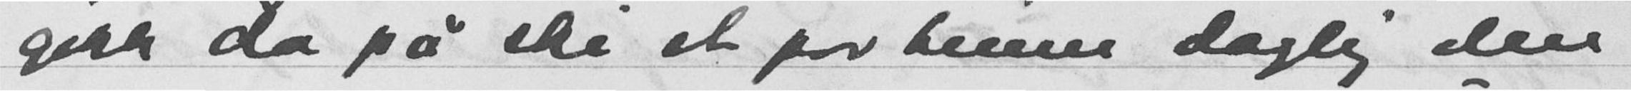gikk da på ski et par timer daglig den
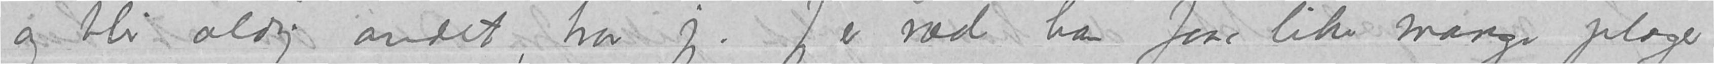og blir aldrig andet, tror jeg. Jeg er ræd han faar like mange plager
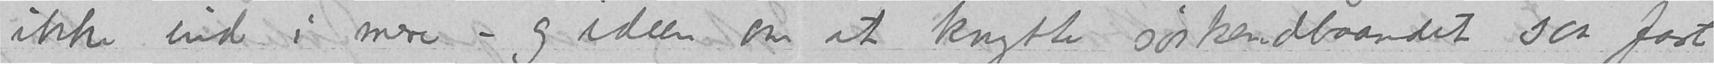ikke ind i mere – og ideen om at knytte søskendbaandet saa fast
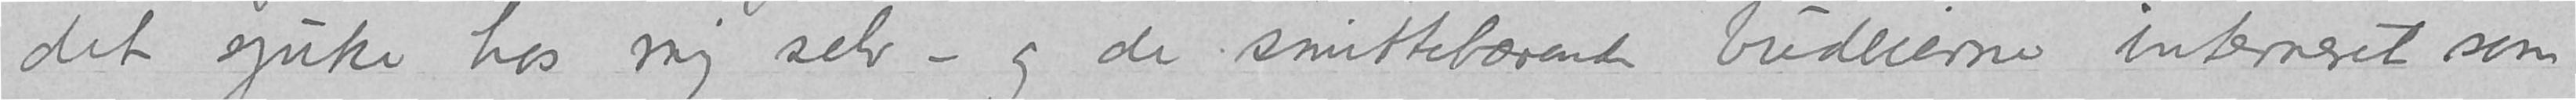det sjuke hos mig selv – og de smittebærende budeierne interneret som
